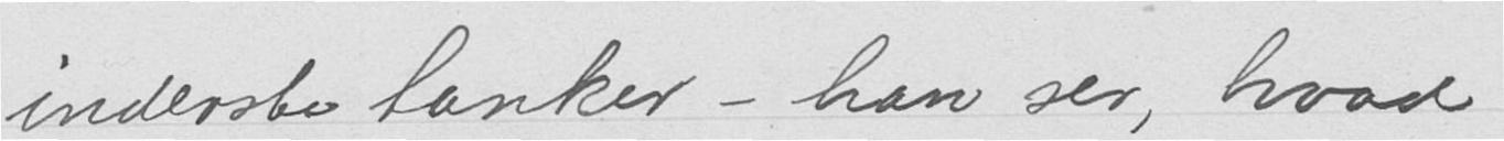inderste tanker – han ser, hvad
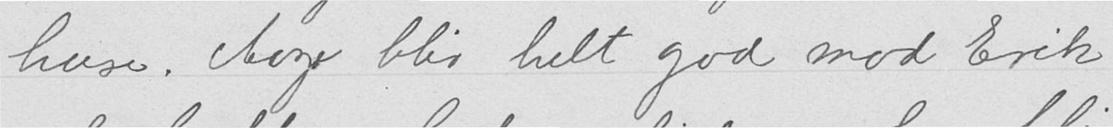huse. Aage blir helt god mod Erik
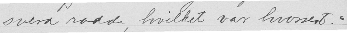sverd raade, hvilket var hvassest."
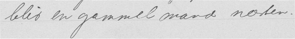blir en gammel mand næsten.
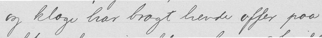og klage har bragt hende offer paa
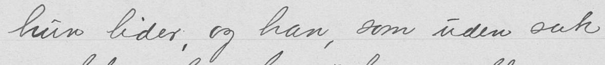hun lider, og han, som uden suk
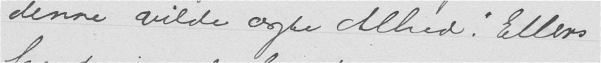denne vilde ægte Alhed. "Ellers
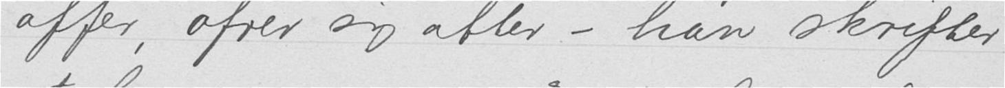offer, ofrer sig atter – han skrifter
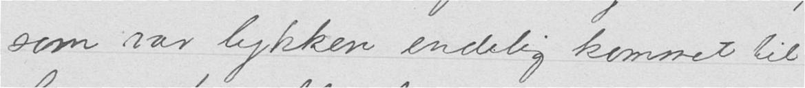som var lykken endelig kommet til
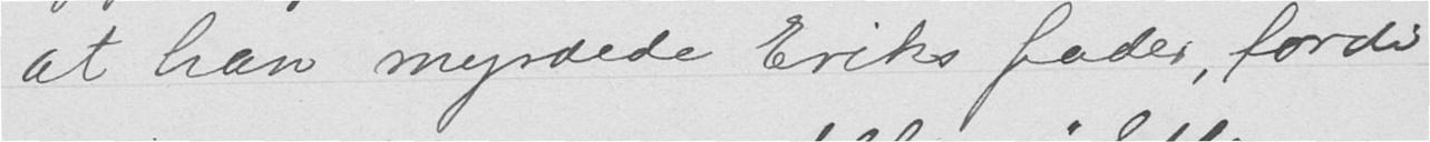at han myrdede Eriks fader, fordi
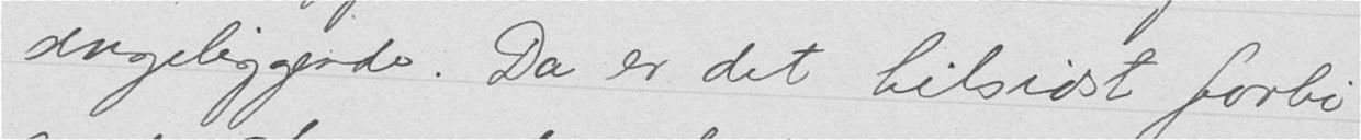sengeliggende. Da er det tilsidst forbi
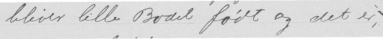bliver lille Bodil født og det er,
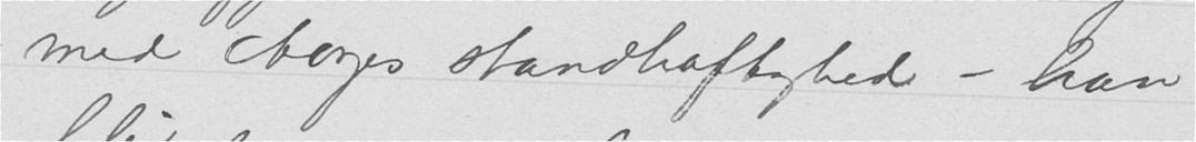med Aages standhaftighed – han
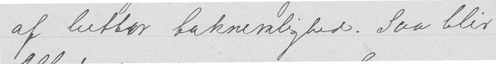af lutter taknemlighed. Saa blir
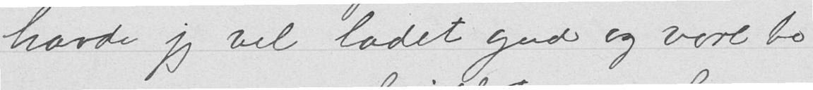havde jeg vel ladet gud og vore to
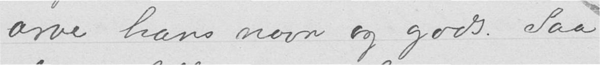arve hans navn og gods. Saa
